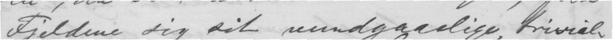Fjeldene sig sit uundgaaelige, triviele
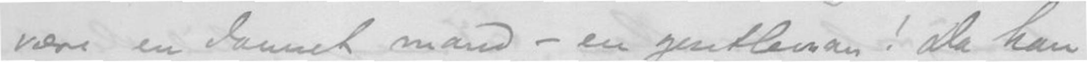være en dannet mand - en gentleman! Da han
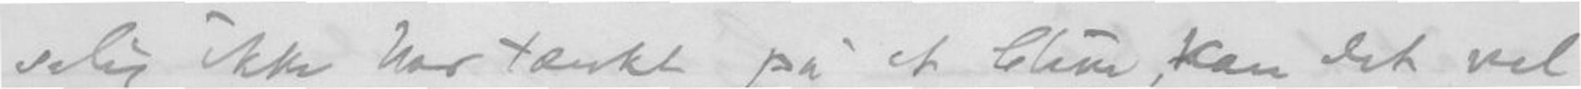selig ikke har tænkt på at blive, kan det vel
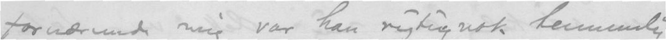fornærmede mig var han rigtignok temmelig
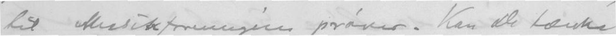til Musikforeningens prøver. Kan De tænke
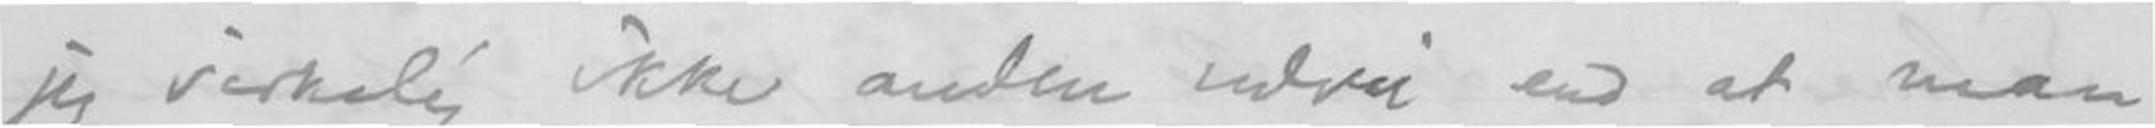jeg virkelig ikke anden udvei end at man
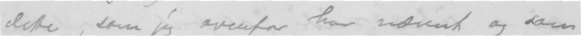dette, som jeg ovenfor har nævnt og som
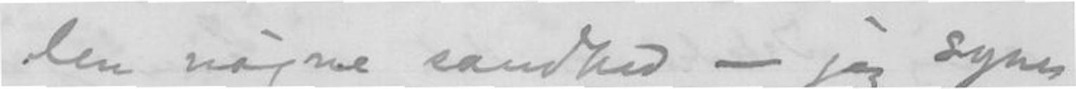den nøgne sandhed - jeg synes
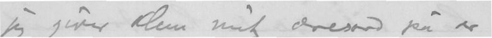jeg giver Dem mit æresord på er
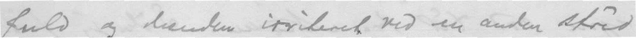fuld og desuden irriteret ved en anden strid
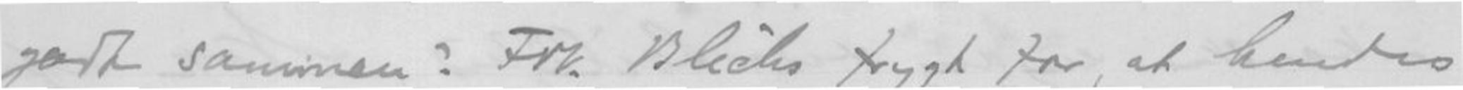godt sammen! Frk Blichs frykt for, at hendes
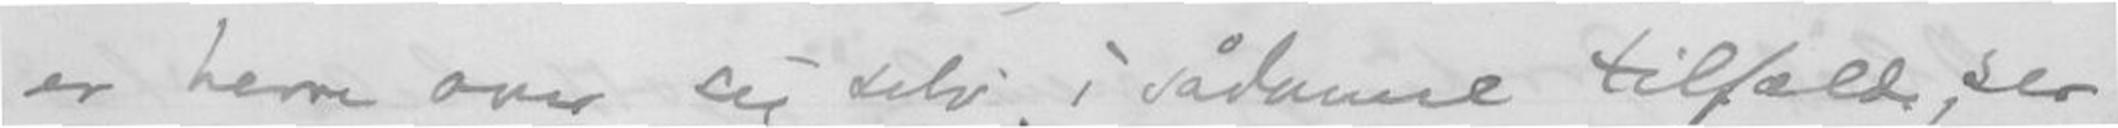er herre over sig selv i sådanne tilfælde, ser
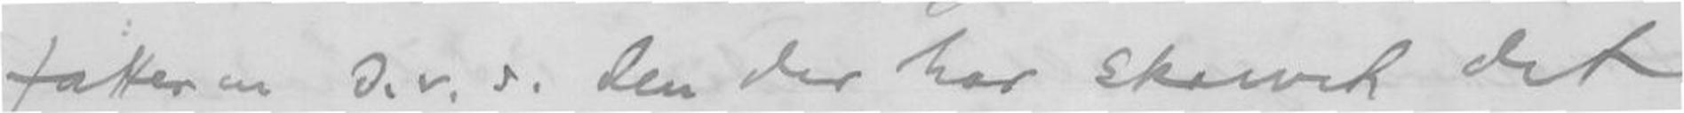fatteren d.v.s. den der har skrevet det
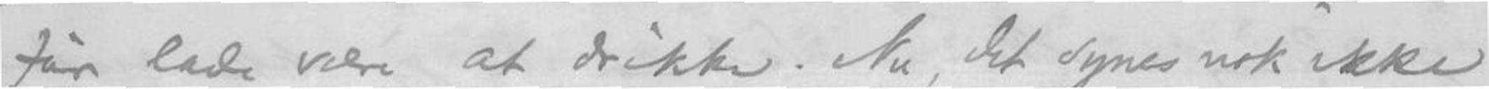får lade være at drikke. Nu, det synes nok ikke
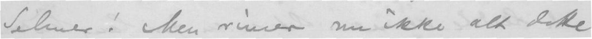Selmer! Men rimer nu ikke alt dette
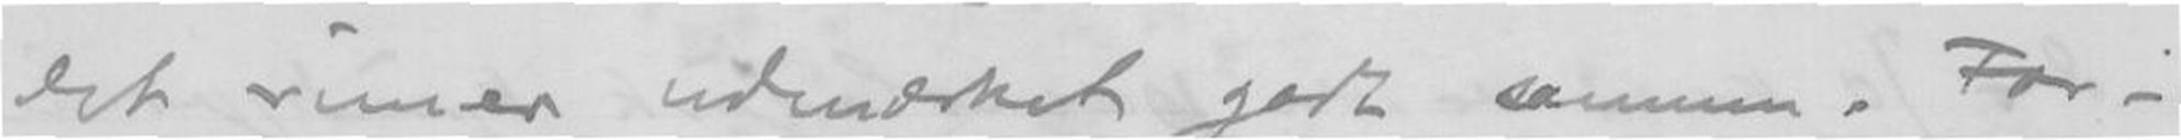det rimer udmærket godt sammen. For-
